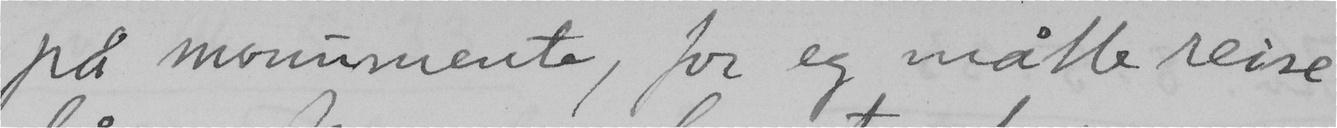på momumente, for eg måtte reise
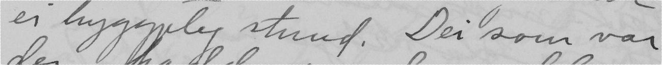er hyggjeleg stund. Dei som var
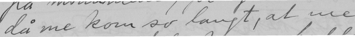då me kom so langt, at me
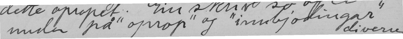under på" "oprop" og "innbjodingar" diverre.
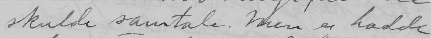skulde samtale. Men eg hadde
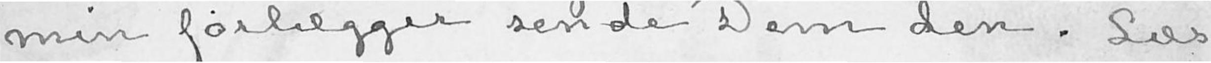min forlægger sende Dem den. Læs
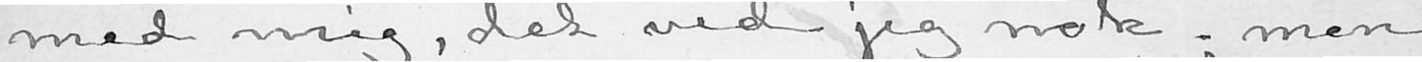med mig, det ved jeg nok; men
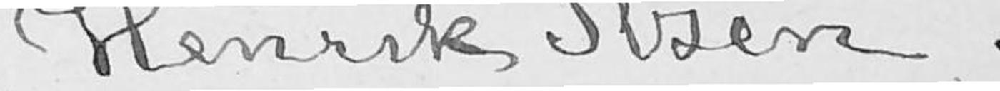Henrik Ibsen.
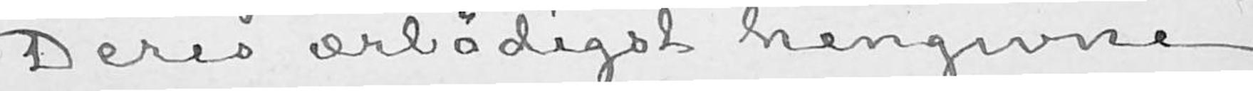Deres ærbødigst hengivne
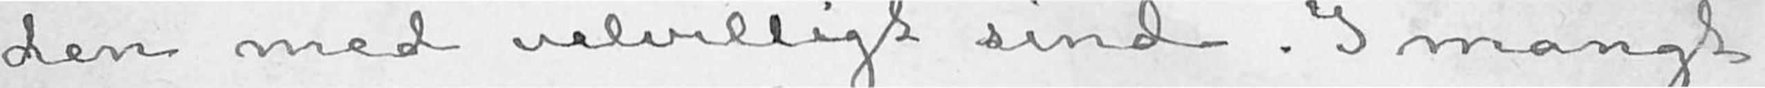den med velvilligt sind. I mangt
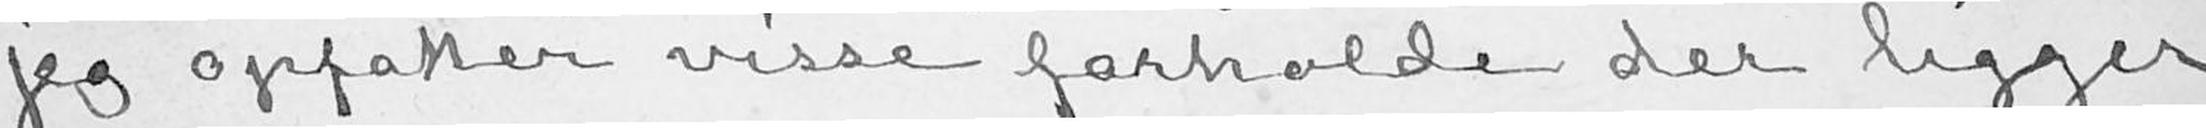jeg opfatter visse forholde der ligger
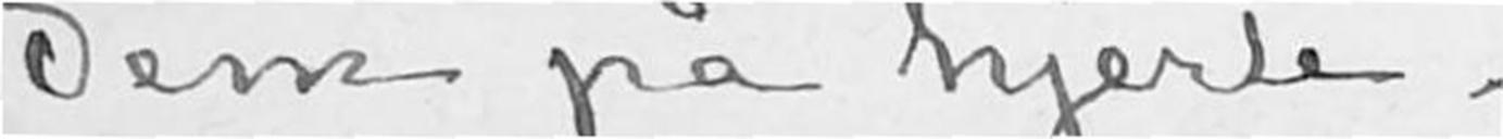Dem på hjerte.
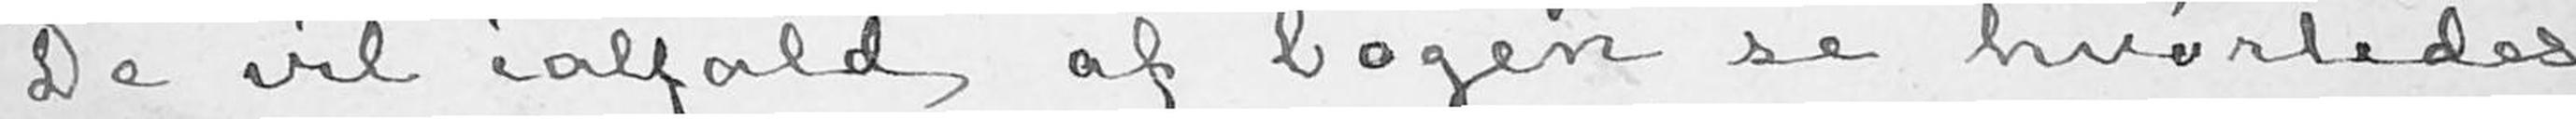De vil ialfald af bogen se hvorledes
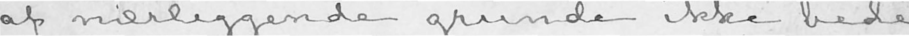af nærliggende grunde ikke bede
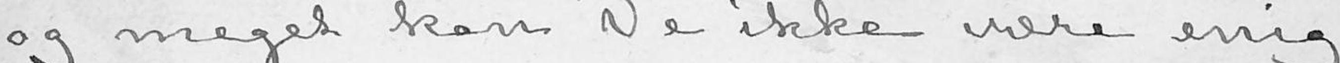og meget kan De ikke være enig
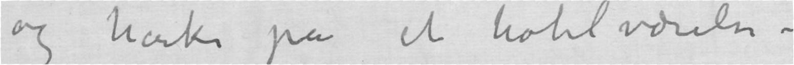og harke på et hotelværelse –
